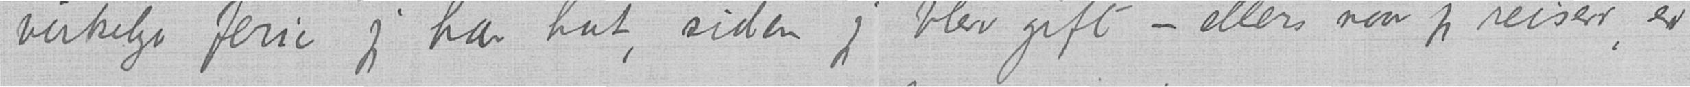virkelige ferie jeg har hat, siden jeg blev gift — ellers naar jeg reiser, er
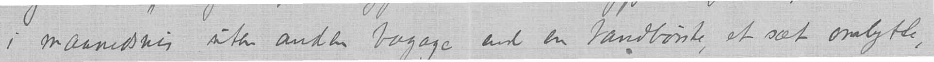i maanedsvis uten anden bagage end en tandbørste, et sæt ombytte,
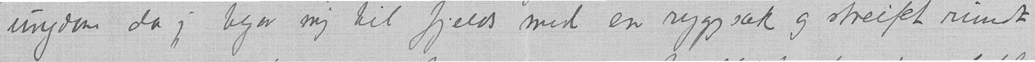ungdom da jeg begav mig til fjelds med en ryggsæk og streifet rundt
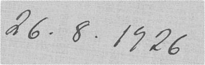26.8.1926
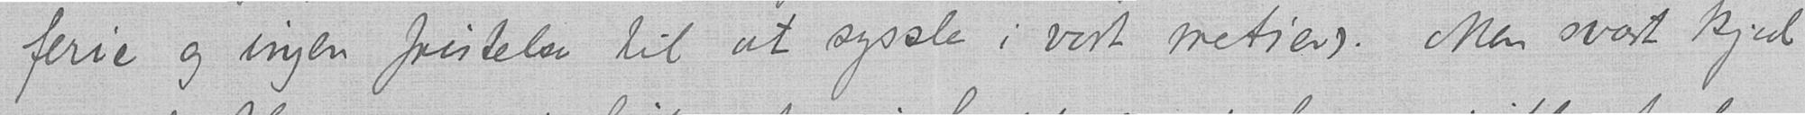ferie og ingen fristelse til at syssle i vort metier). Men svært kjed
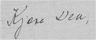Kjære Dea,
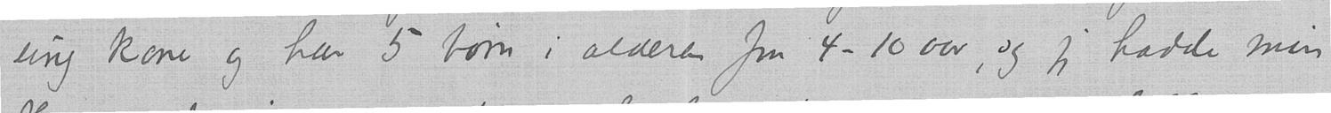ung kone og har 5 børn i alderen fra 4-10 aar, og jeg hadde min
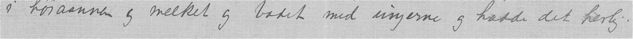i høionnen og melket og badet med ungerne og hadde det herlig.
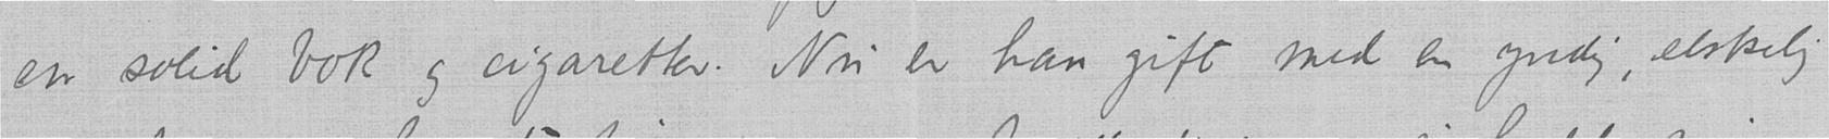en solid bok og cigaretter. Nu er han gift med en yndig, elskelig
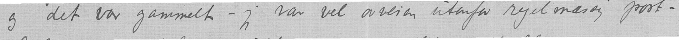og det var gammelt — jeg var vel avveien utenfor regelmæssig post-
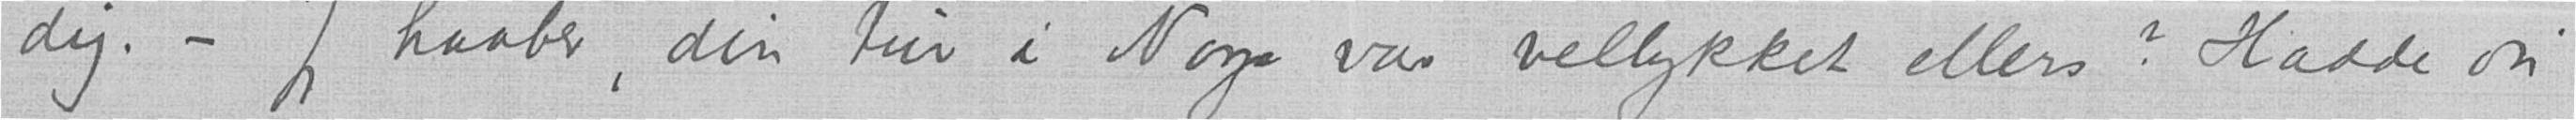dig. — Jeg haaber, din tur i Norge var vellykket ellers? Hadde du
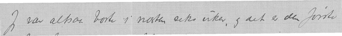Jeg var altsaa borte i næsten seks uker, og det er den første
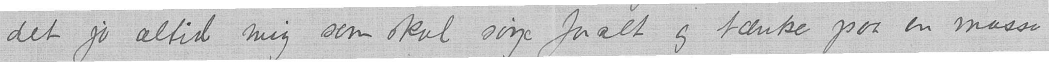det jo altid mig som skal sørge for alt og tænke paa en masse
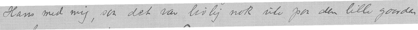Hans med mig, saa det var livlig nok ute paa den lille gaarden
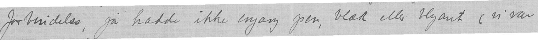forbindelse, ja hadde ikke engang pen, blæk eller blyant (vi var
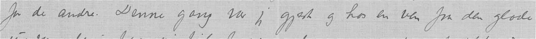for de andre. Denne gang var jeg gjest og hos en ven fra den glade
